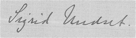Sigrid Undset.
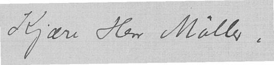Kjære Herr Møller,
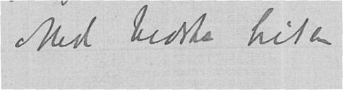Med bedste hilsen
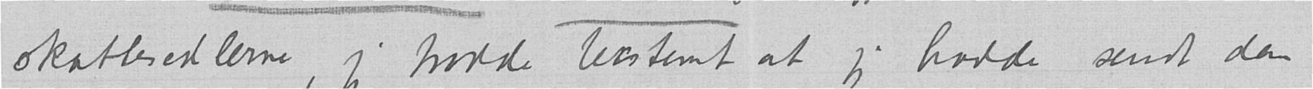skattesedlerne, jeg trodde bestemt at jeg hadde sendt dem
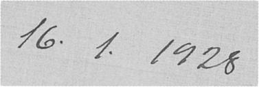16.1.1928
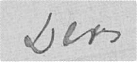Deres
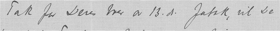Tak for Deres brev av 13.ds. Jatak, vil De
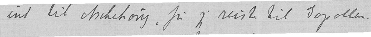ind til Aschehoug, før jeg reiste til Gopollen.
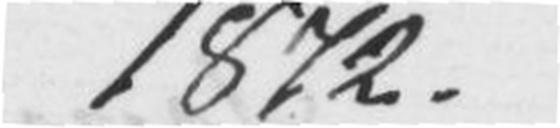1872.
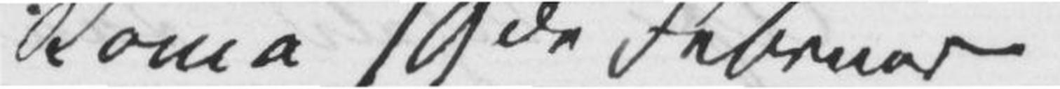Roma 19de Februar
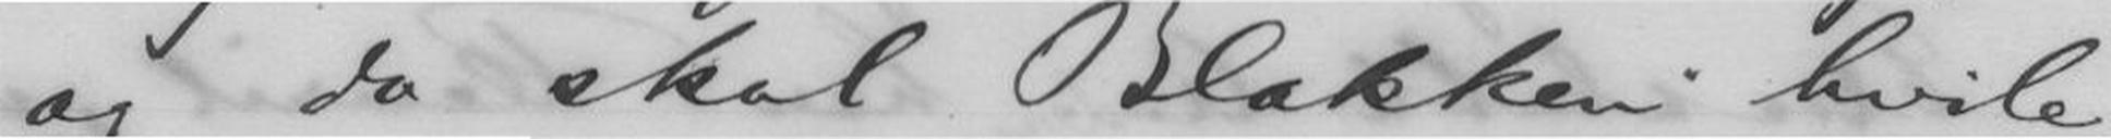og da skal Blakken hvile,
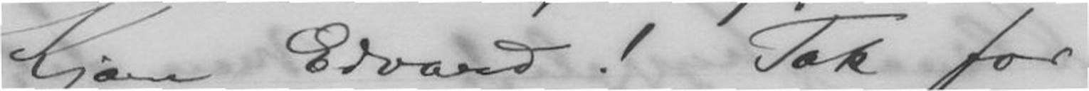Kjære Edvard!Tak for
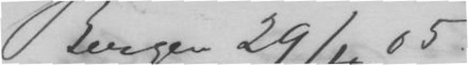Bergen 29/4 05
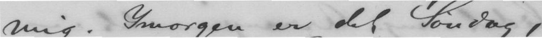mig. Imorgen er det Søndag,
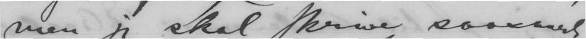men jeg skal skrive saasnart
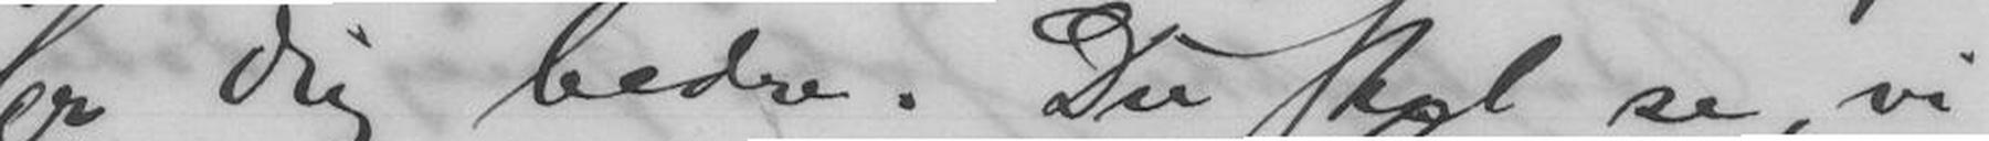ler dig bedre. Du skal se, vi
